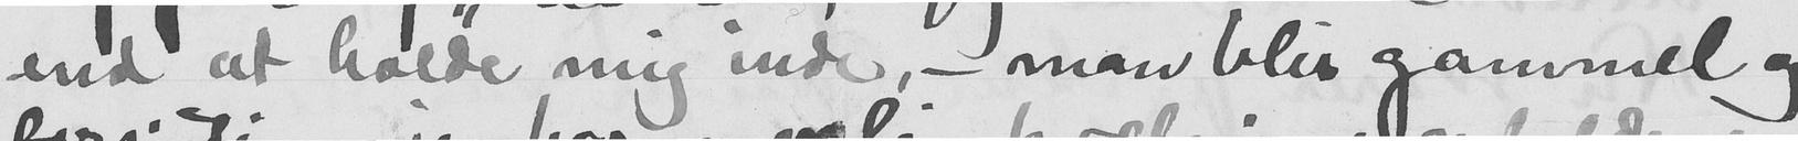end at holde mig inde, - man blir gammel og
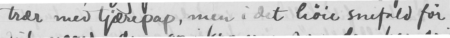trær med tjærepap, men i det høie snefald før
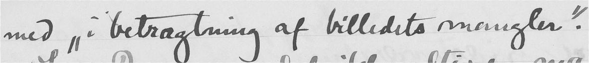med "i betragtning af billedets mangler".
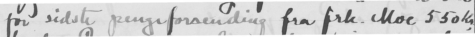for sidste pengeforsending fra frk. Moe 550 Kr.,
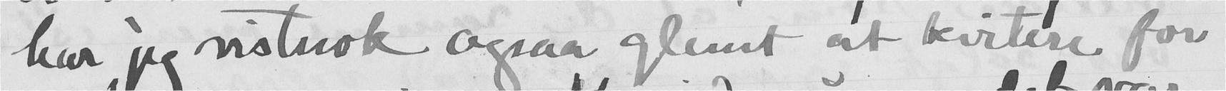har jeg vistnok ogsaa glemt at kvitere for
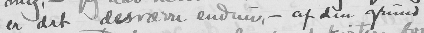er det desværre endnu, - af den grund
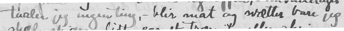taaler jeg ingenting, - blir mat og svætter bare jeg
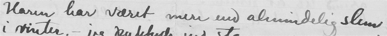Haren har været mere end almindelig slem
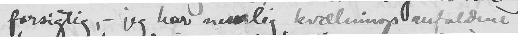forsigtig, - jeg har nemlig kvælningsanfaldene
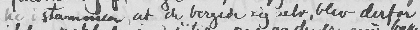ke i stammen, at de bergede sig selv, blev derfor
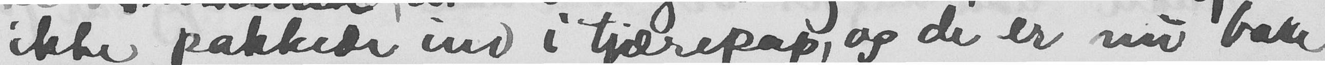ikke pakkede ind i tjærepap, og de er nu bare
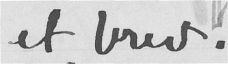et brev.
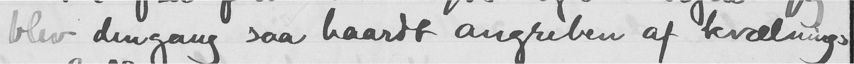blev dengang saa haardt angreben af kvælnings
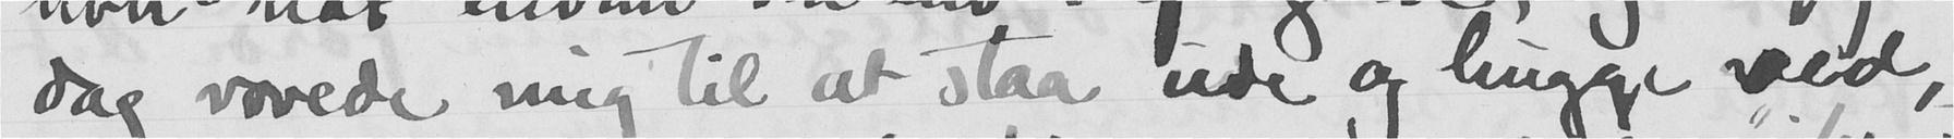dag vovede mig til at staa ude og hugge ved,
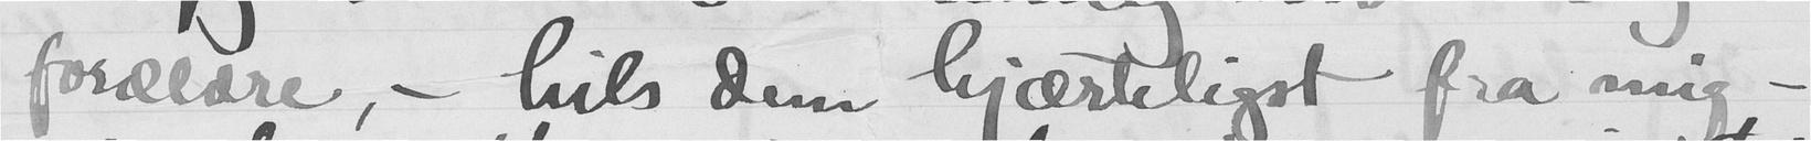forældre, - hils dem hjærteligst fra mig -
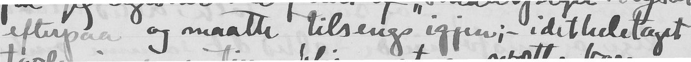efterpaa og maatte tilsengs igjen; - i det hele taget
